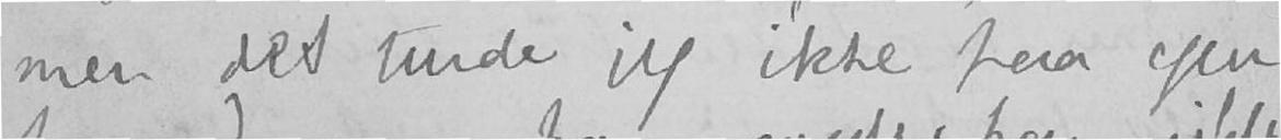men det turde jeg ikke paa egen
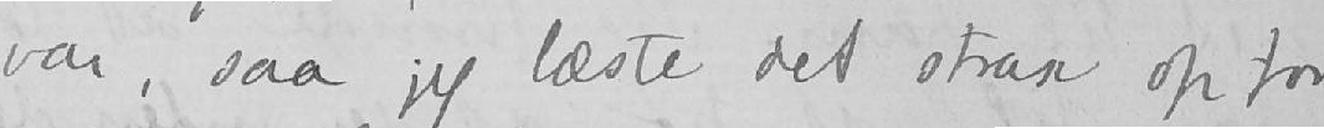var, saa jeg læste det strax op for
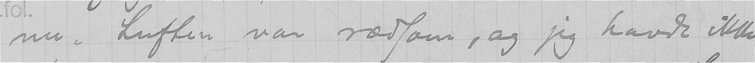nu. Luften var rædsom, og jeg havde ikke
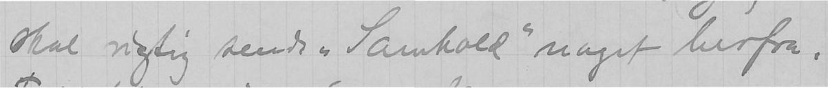skal rigtig sende "Samhold" noget herfra.
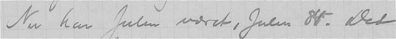Nu har Julen været, Julen 84. Det
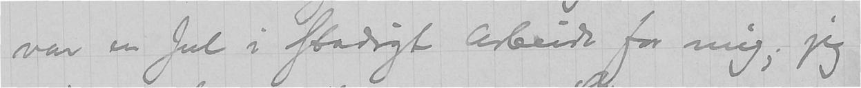var en Jul i stadigt Arbeide for mig; jeg
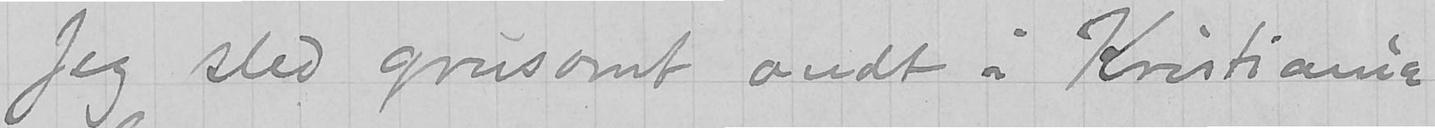Jeg sled grusomt ondt i Kristiania
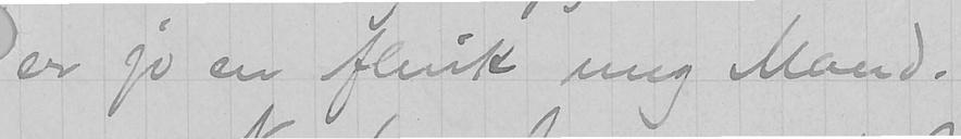er jo en flink ung Mand.
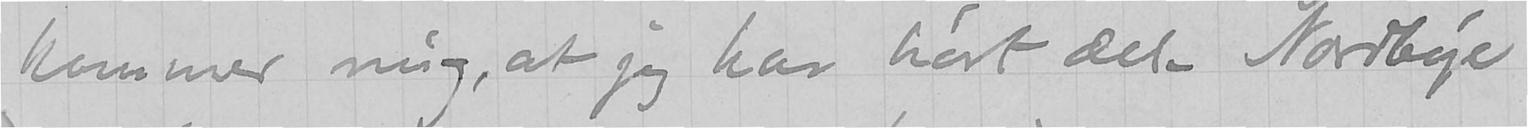kommer mig, at jeg har hørt det. Nordbye
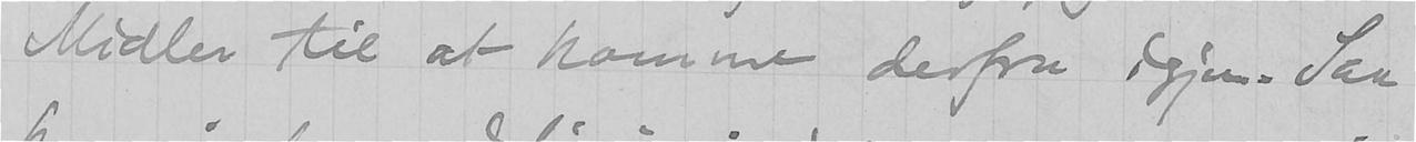Midler til at komme derfra igjen. Saa
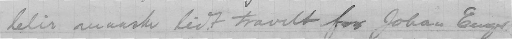blir maaske lidt travelt for Johan Enger
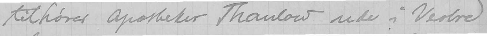tilhører Apotheker Thaulow ude i Vestre
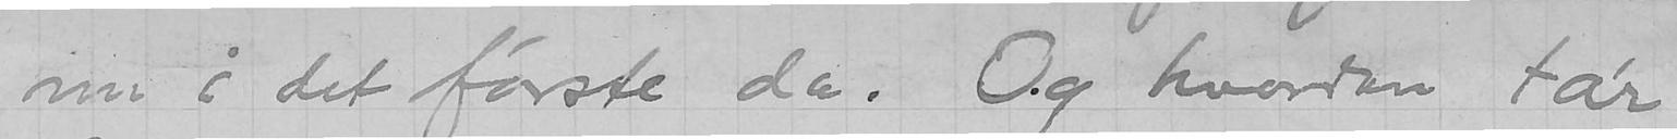nu i det første da. Og hvordan tar
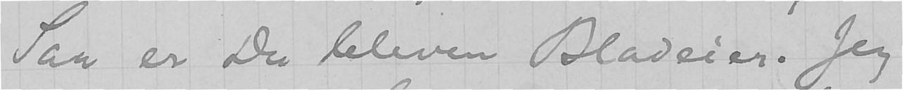Saa er Du bleven Bladeier. Jeg
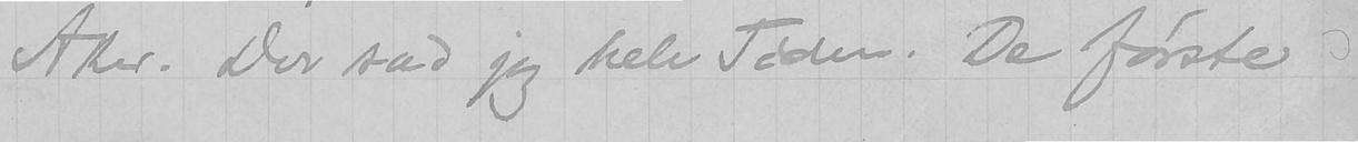Aker. Der sad jeg hele Tiden. De første
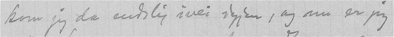kom jeg da endelig ivei igjen, og nu er jeg
